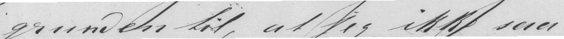grunden til, at jeg ikke saa
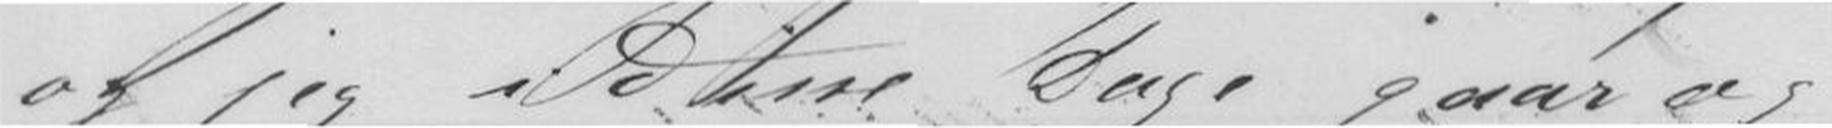og jeg i disse Dage, gaar og
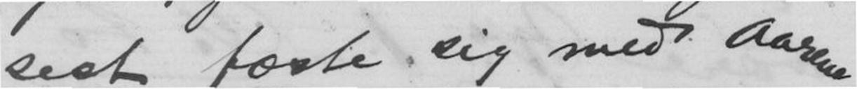seet fæste sig med Aarene,
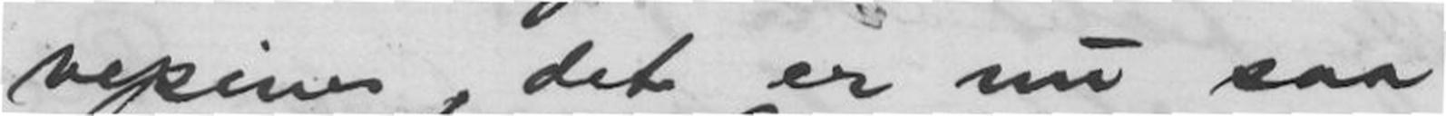vepine, det er nu saa
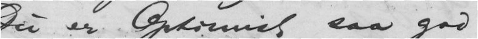Du er Optimist saa god
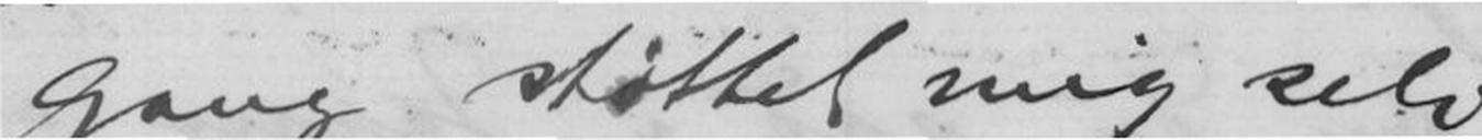Gang støttet mig selv.
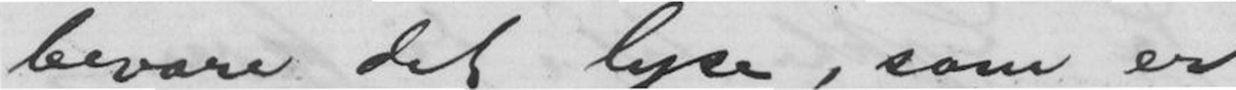bevare det lyse, som er
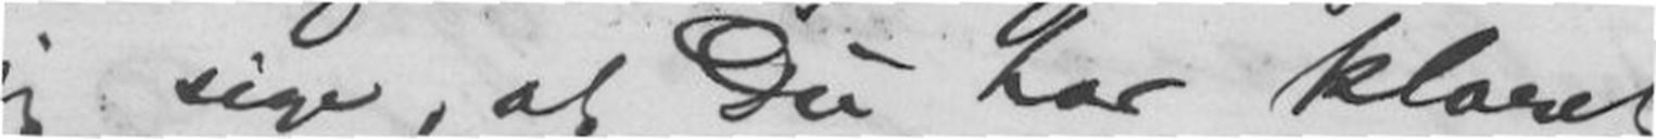jeg sige, at Du har klaret
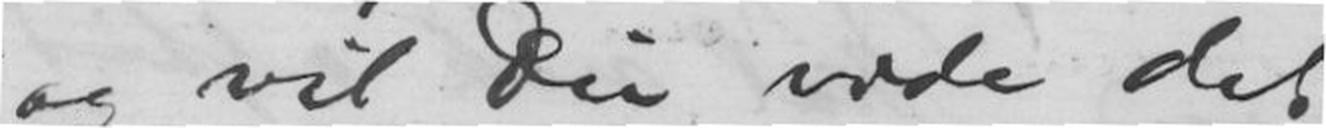og vil Du vide det,
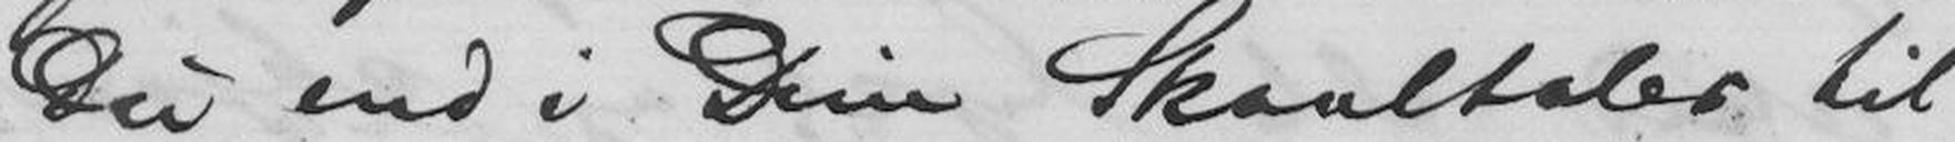Du end i Dine Skaaltaler til
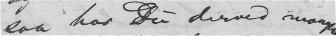saa har Du derved mangen
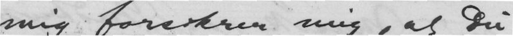mig forsikrer mig, at Du
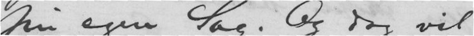sin egen Sag. Og dog vil
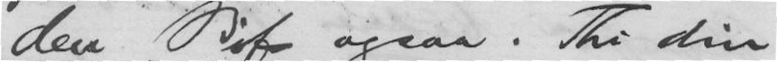den Pif ogsaa. Thi din
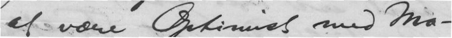at være Optimist med Ma-
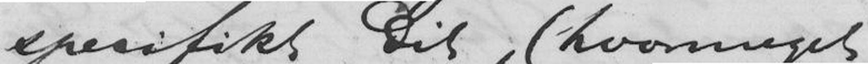spesifikt Dit, (hvormeget
